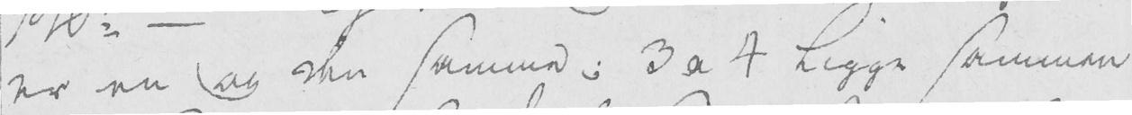er en og den samme; 3 a 4 ligge sammen
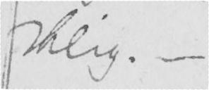delig.
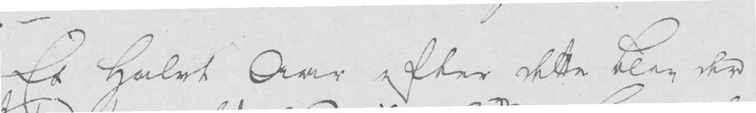Et Halvt Aar efter dette blev der
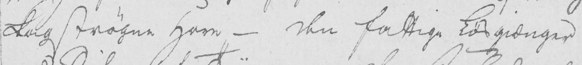kagstrøgne Hore. Den fattige Løsgiænger
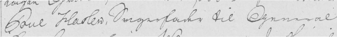Poul Haslev, Svigerfader til General
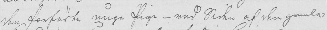den forførte unge Pige - ved Siden af den gamle
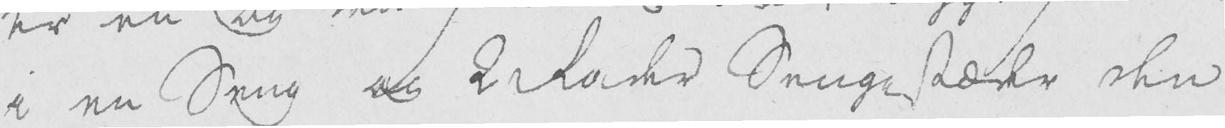i en Seng og 2 Rader Sengestæder den
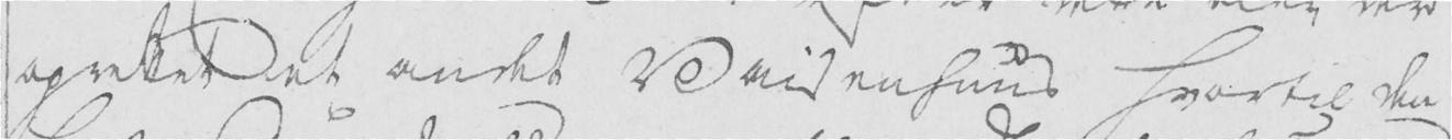oprettet et andet Vaisenhuus hvortil den
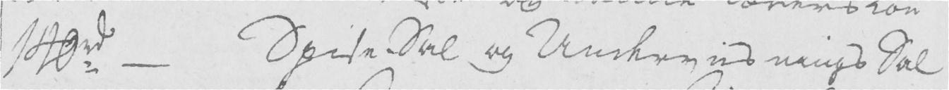140 rd - Spise-Sal og Undervisnings Sal
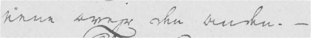eene over den anden. -
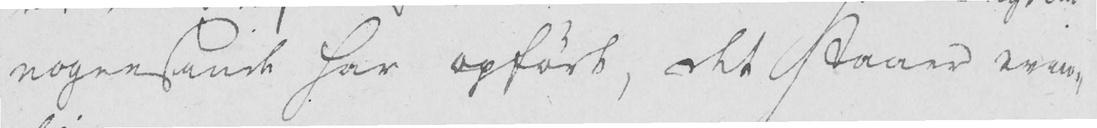nogensinde har opført, det staaer sviv-
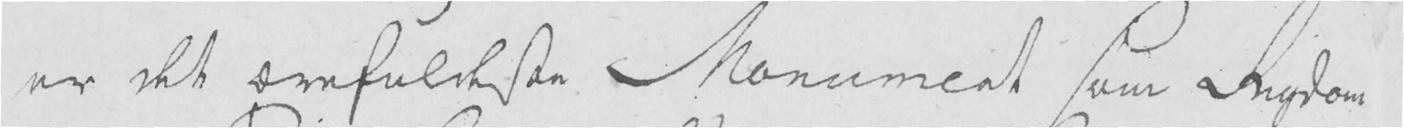er det ærefuldeste Monument som Rigdom
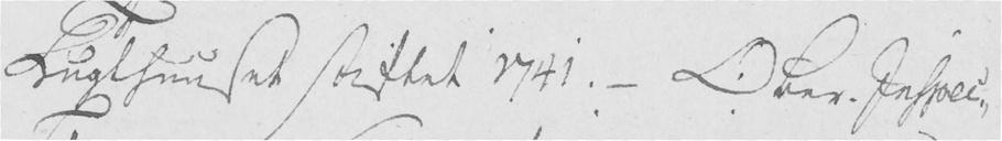Tugthuuset stiftet 1741. - Ober-Inspec-
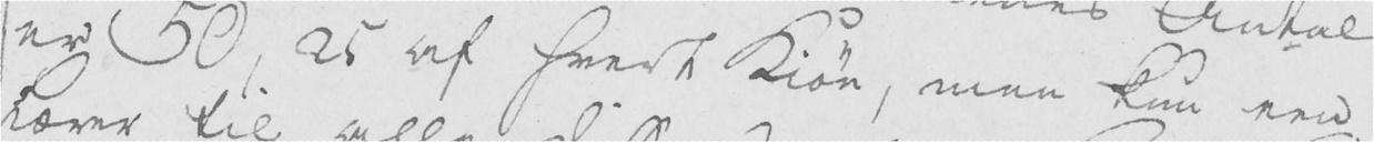er 50, 25 af hvert Kiøn, men kun een
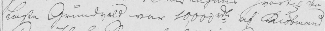lagte Grundvold var 10,000 rde af Kiøbmand
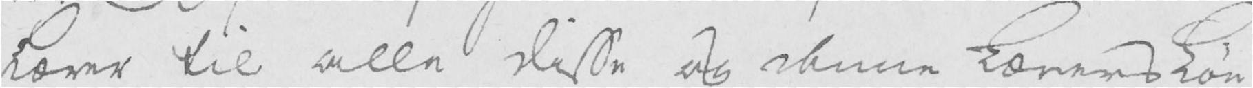Lærer til alle disse og denne Lærers Løn
